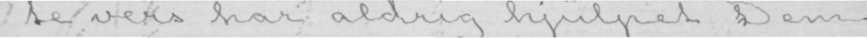te vers har aldrig hjulpet Dem
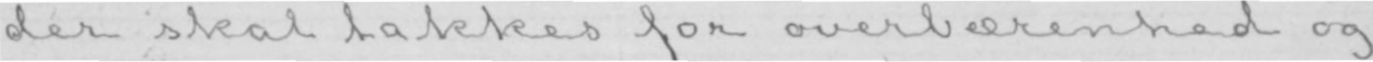der skal takkes for overbærenhed og
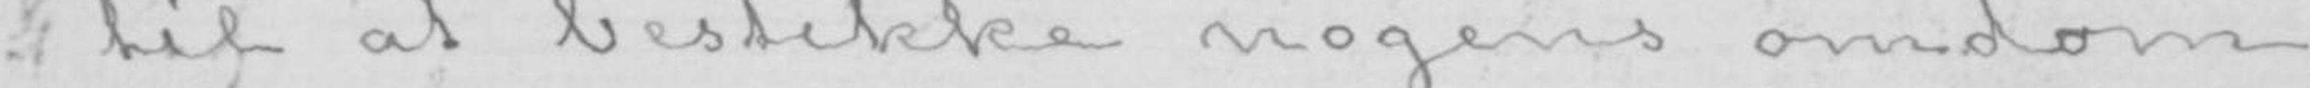til at bestikke nogens omdøm-
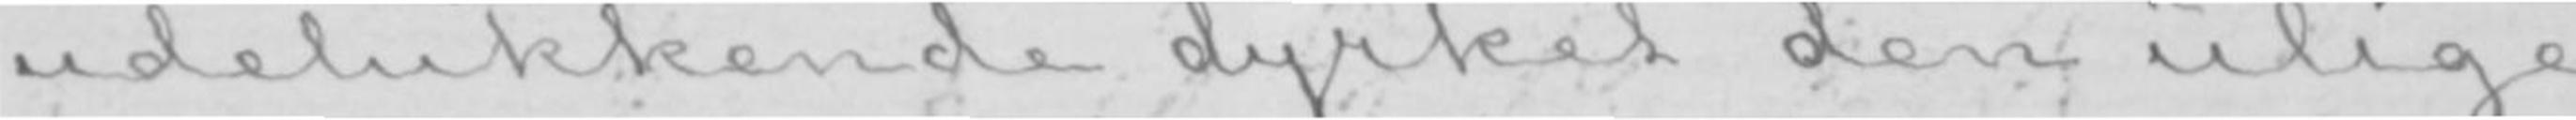udelukkende dyrket den ulige
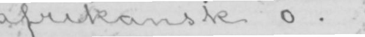afrikansk ø.
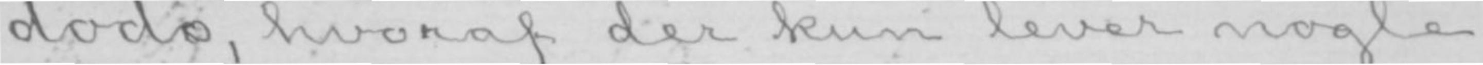dodo, hvoraf der kun lever nogle
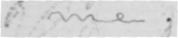me.
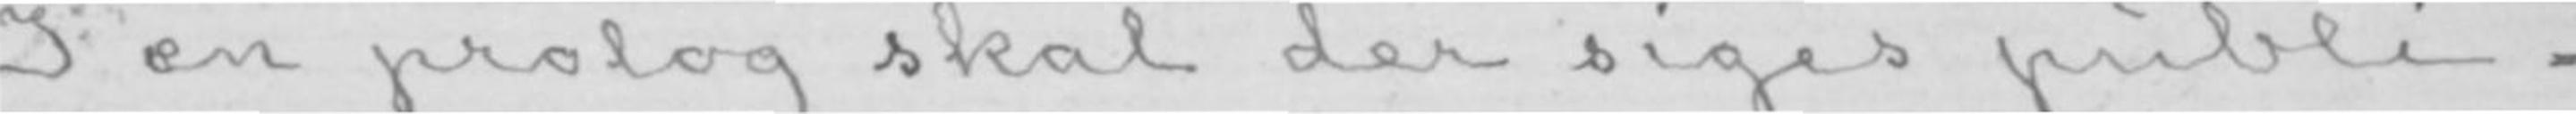I en prolog skal der siges publi-
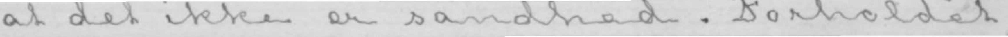at det ikke er sandhed. Forholdet
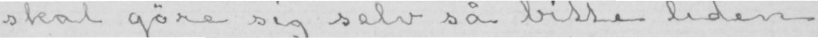skal gøre sig selv så bitte liden,
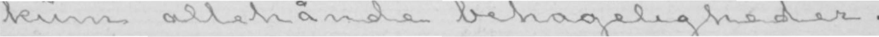kum allehånde behageligheder;
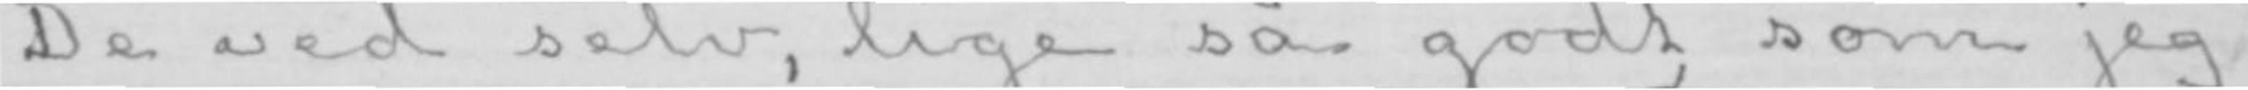De ved selv, lige så godt som jeg,
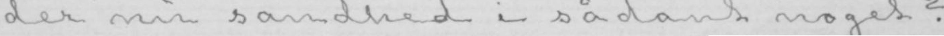der nu sandhed i sådant noget?
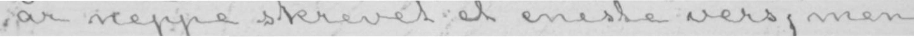år neppe skrevet et eneste vers, men
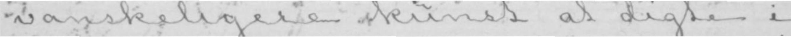vanskeligere kunst at digte i
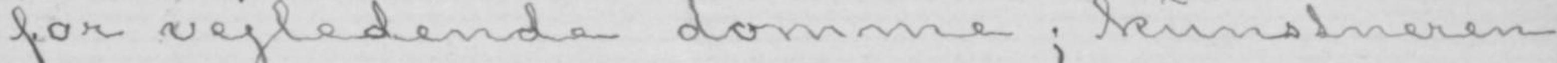for vejledende domme; kunstneren
